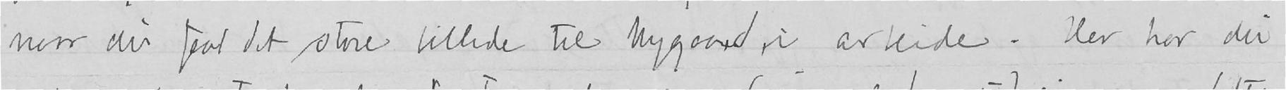naar du faat det store billede til Nygaard i arbeide. Her har du
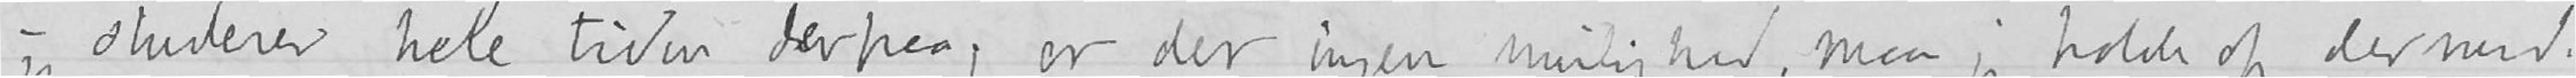jeg studerer hele tiden derpaa; er der ingen mulighed, maa jeg holde op dermed.
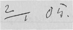2/1 05.
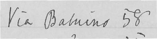Via Babuino 58
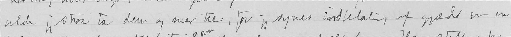vilde jeg strax ta dem og mer til, for jeg synes indbetaling af gjæld er en
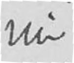nu
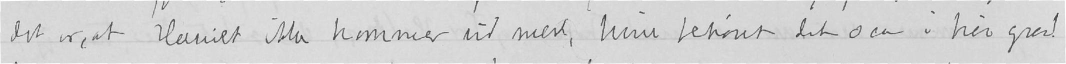det er, at Harriet ikke kommer ud mer, hun behøver det saa i høi grad.
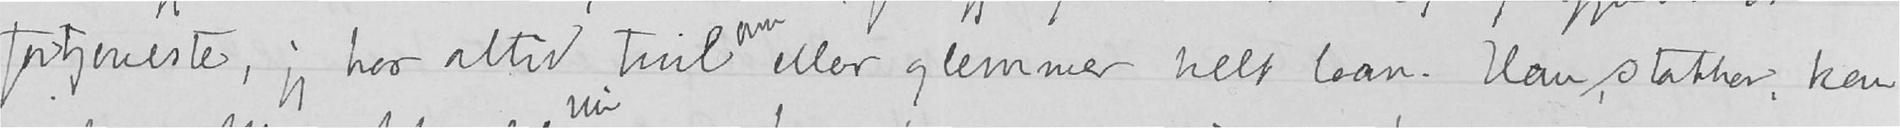fortjeneste, jeg har altid tvivl om eller glemmer helt laan. Han, stakker, kan
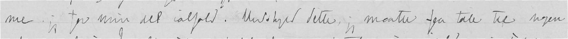me, jeg for min del ialfald. Undskyld dette, jeg maatte faa tale til nogen
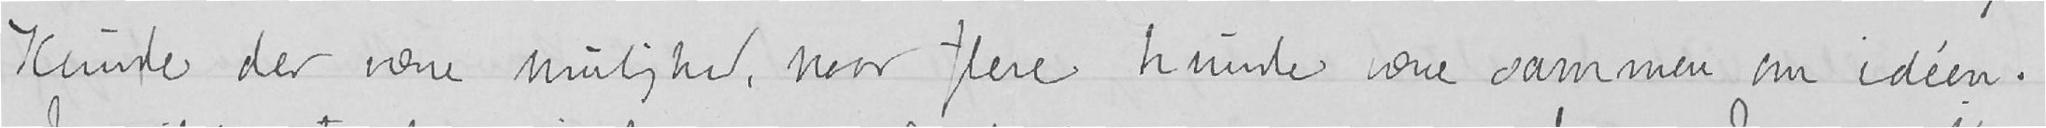Kunde der være mulighed, naar flere kunde være sammen om idéen.
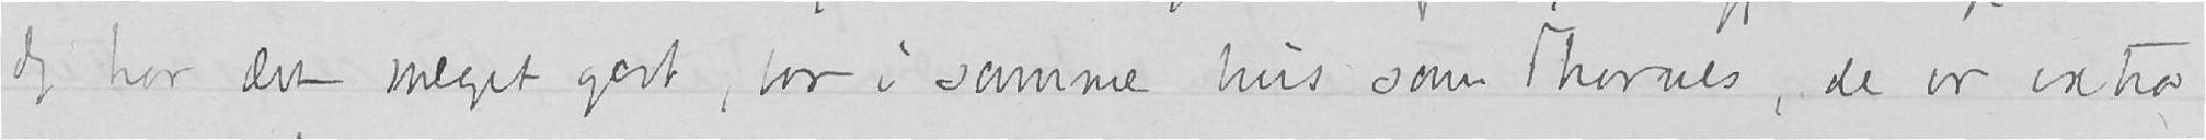Jeg har det meget godt, bor i samme hus som Thornes, de er extra
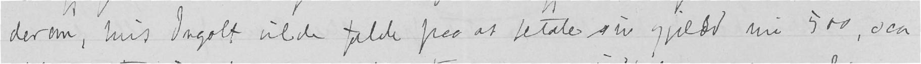derom, hvis Ingolf vilde falde paa at betale sin gjæld nu 500, saa
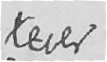lever
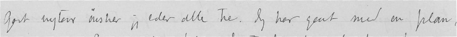Godt nytaar ønsker jeg eder alle tre. Jeg har gaat med en plan,
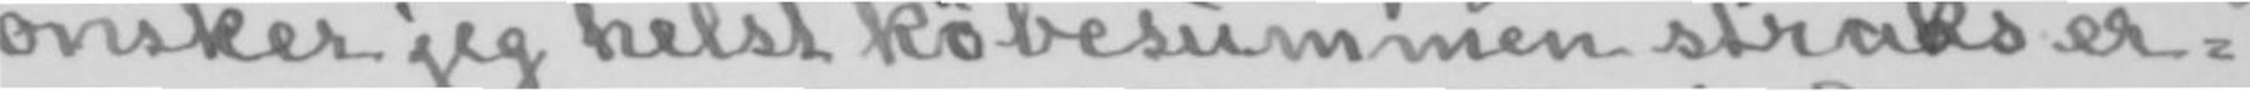ønsker jeg helst købesummen straks er-
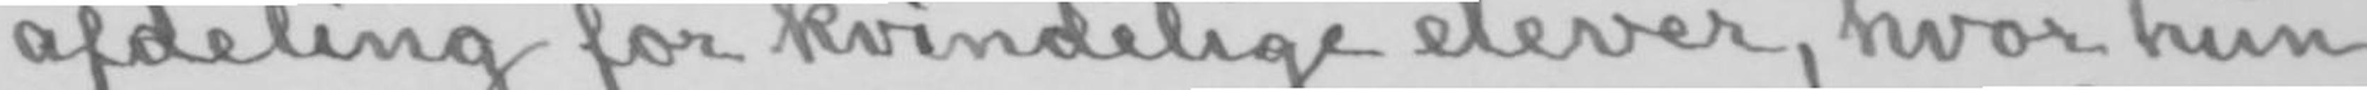afdeling for kvindelige elever, hvor hun
